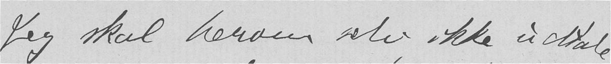Jeg skal herom selv ikke udtale
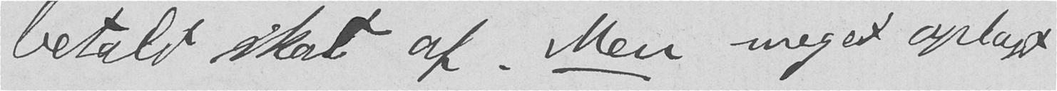betalt skat af. Men meget oplagt
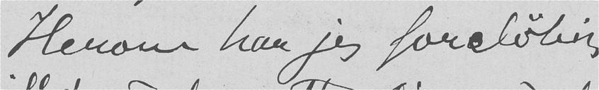Herom har jeg foreløbig
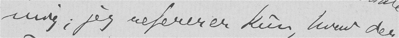mig; jeg refererer kun, hvad der
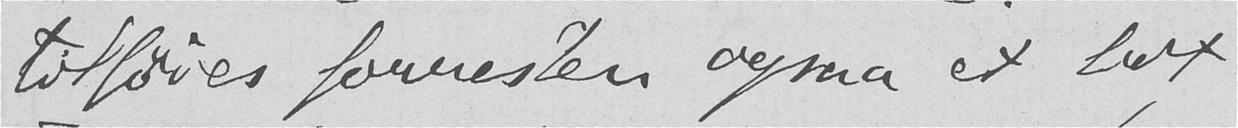tilføies forresten ogsaa et lidt
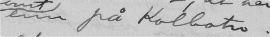enn på Kolbotn.
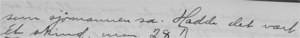som sjømannen sa. Hadde det vært
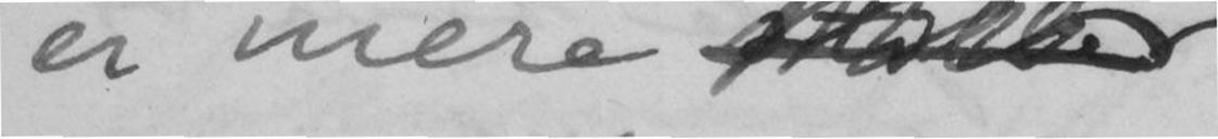er mere
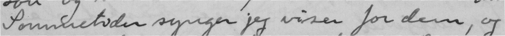Sommetider synger jeg viser for dem, og
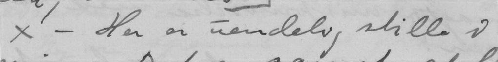x — Her er uendelig stille i
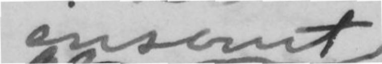ensomt
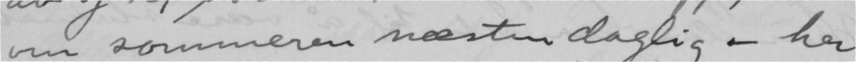om sommeren nesten daglig - her
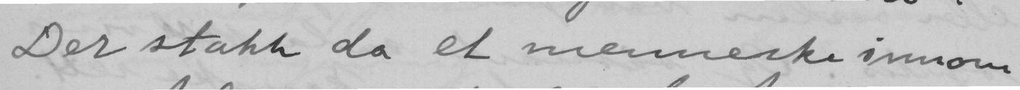Der stakk da et menneske innom
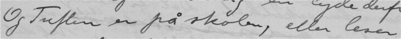Og Tuften er på skolen, eller leser
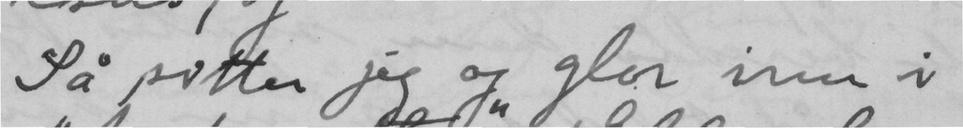Så sitter jeg og glor inn i
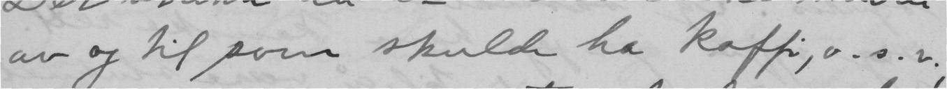av og til som skulde ha kaffi, o.s.v.,
